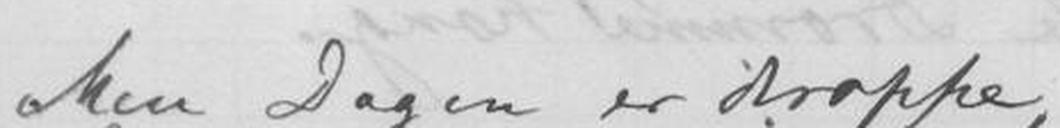Men Dagen er deroppe,
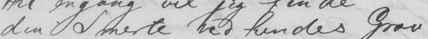den Smerte ved hendes Grav.
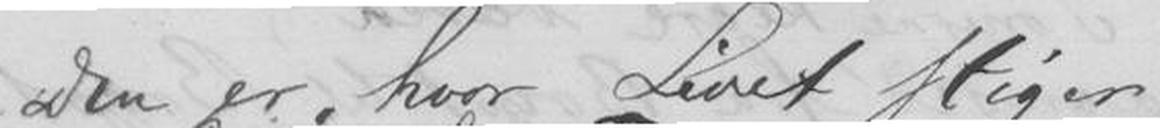Den er, hvor Livet stiger
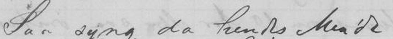Saa syng da hendes Minde
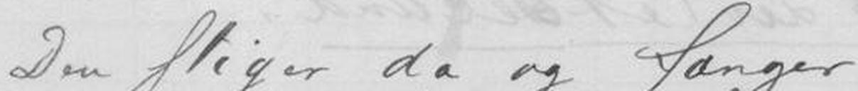Den stiger da og fanger
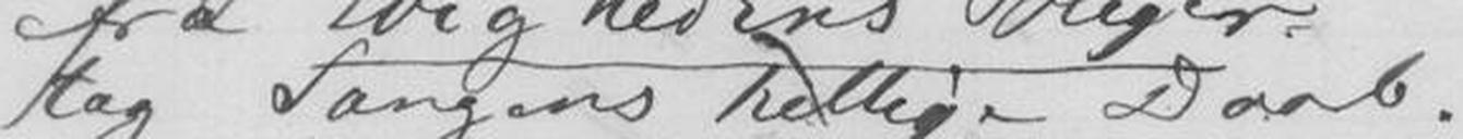tag Sangens hellige Daab.
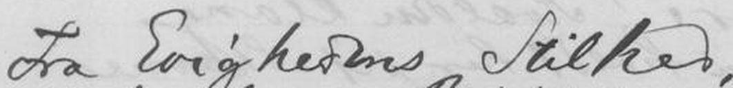Fra Evighedens Stilhed,
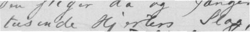tusende Hjerters Slag,
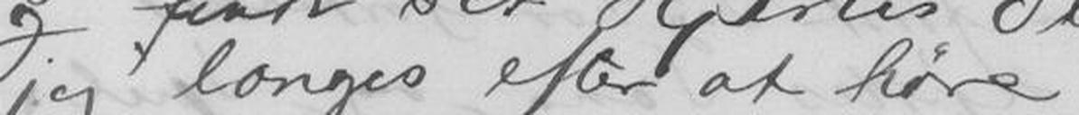jeg længes efter at høre
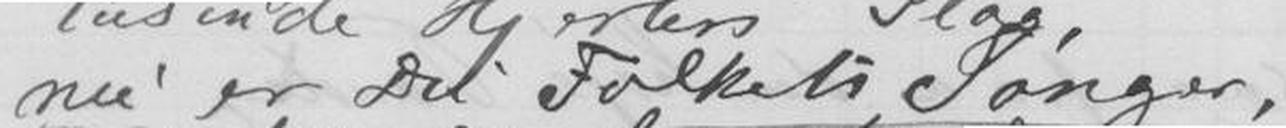nu er Dit Folkets Sanger,
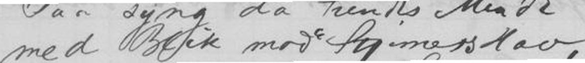med Blik mod Stjernens Hav,
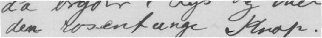den Rosentunge Knop.
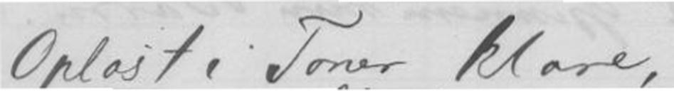Opløst i Toner Klare,
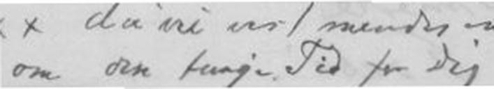om den tunge Tid for dig
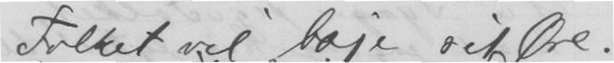Folket vil bøje dit Øre.
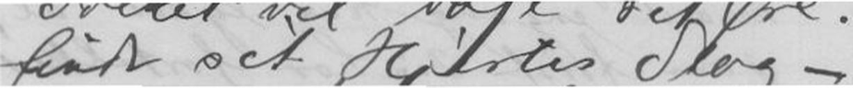og findt sit Hjertes Slog -
